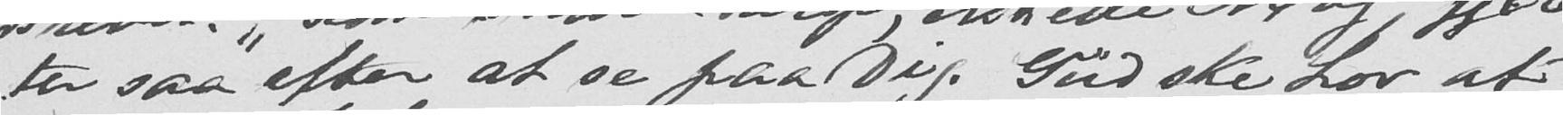ter saa efter at se paa Dig. Gud ske Lov at
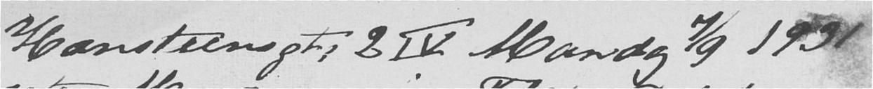Hansteensgt: 2 IV Mandag 7/9 1931
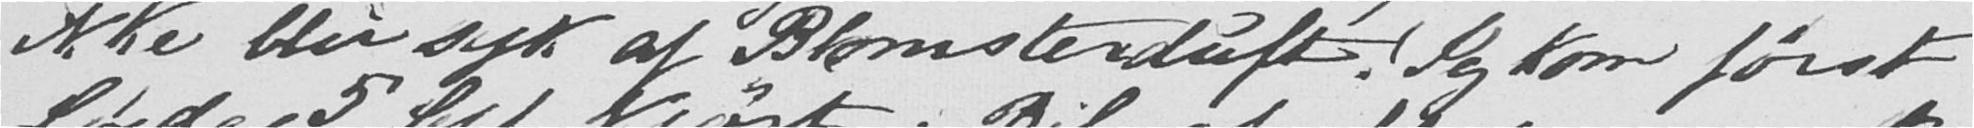ikke blir syk af Blomsterduft! Jeg kom først
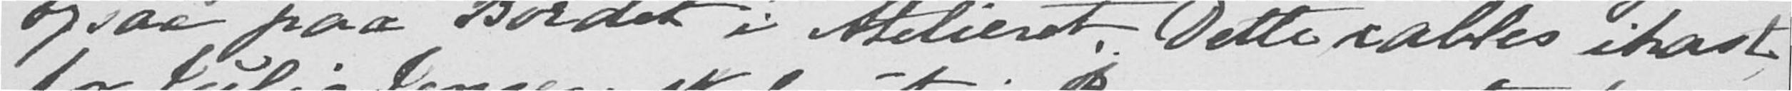ogsaa paa Bordet i Atelieret. Dette rables ihast
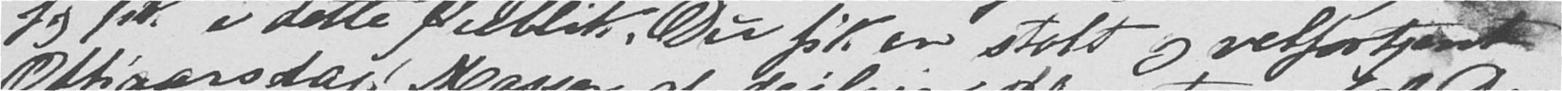jeg fik i dette Øieblik. Du fik en stolt og velfortjent
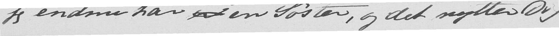jeg endnu har et en Søster, og det nytter Dig
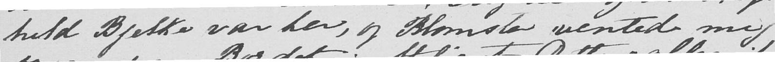hild Bjelke var her, og Blomster ventede mig
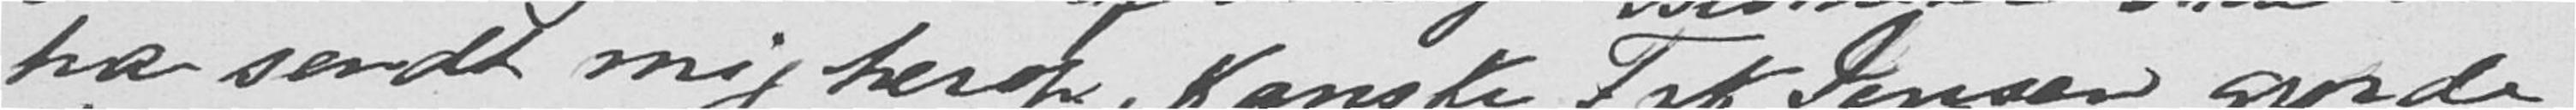ha sendt mig herop, kanske Frk Jensen gjorde
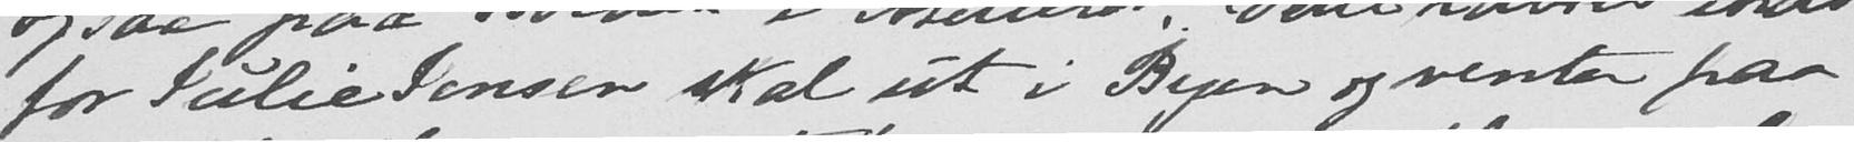for Julie Jensen skal ut i Byen og venter paa
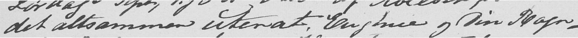det altsammen utenat. Eugenie og Din Ragn-
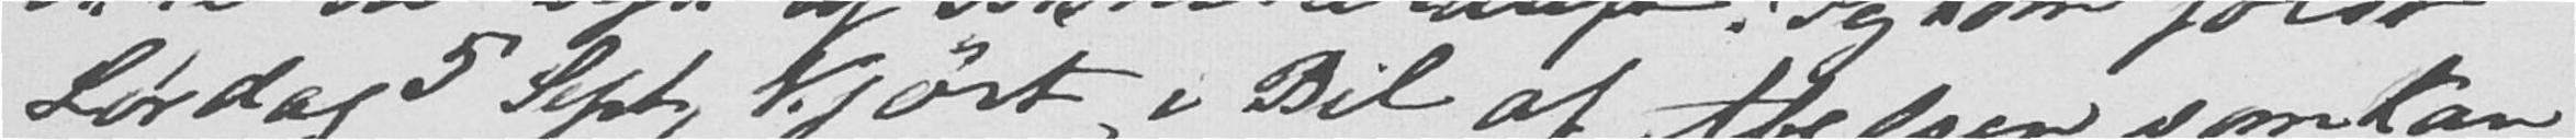Lørdag 5 Sept, kjørt i Bil af Abelsen, som kan
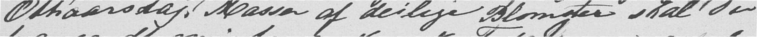Ottiaarsdag. Masser af deilige Blomster skal Du
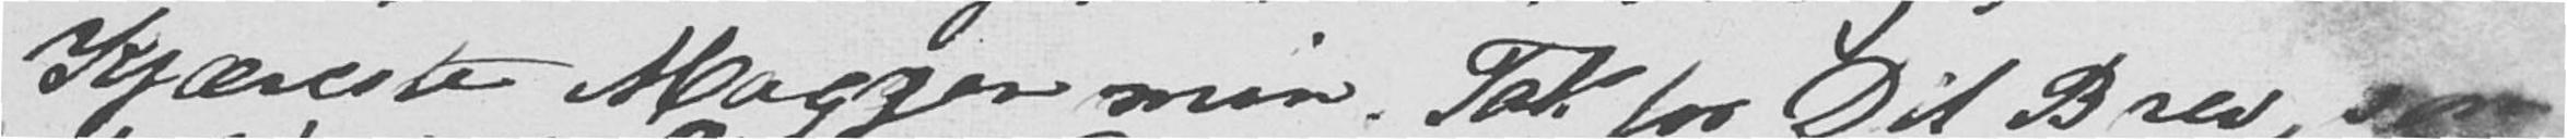Kjæreste Maggen min. Tak for Dit Brev, som
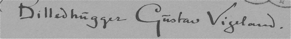Billedhugger Gustav Vigeland.
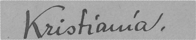Kristiania.
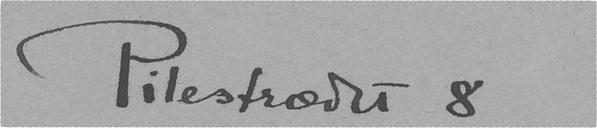Pilestrædet 8
